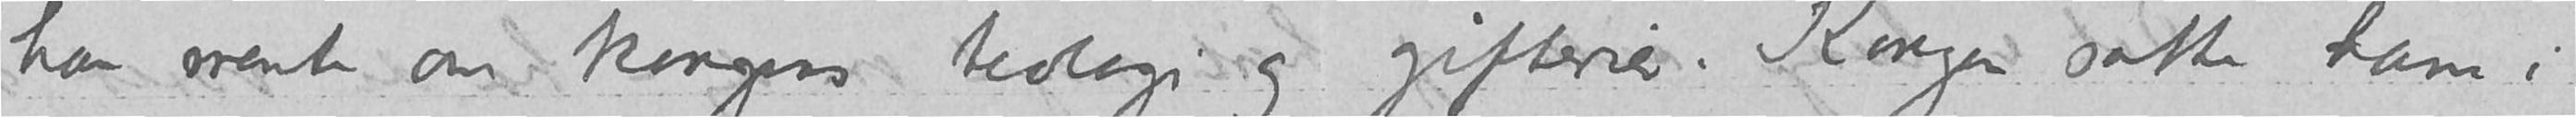han  mente om kongens teologi og gifterier. Kongen satte  ham i
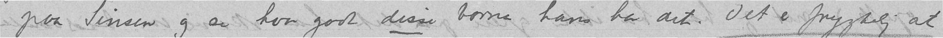paa Sinsen og se hvor godt disse barna hans har det. Det er frygtelig at
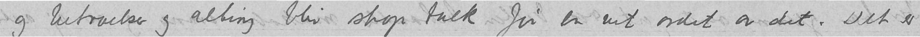og betroelser og alting blir shop talk før en vet ordet av det. Det er
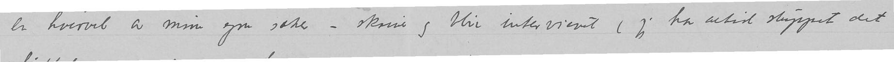en hvirvel av mine egne saker – skriver og blir intervievit (jeg har altid sluppet det
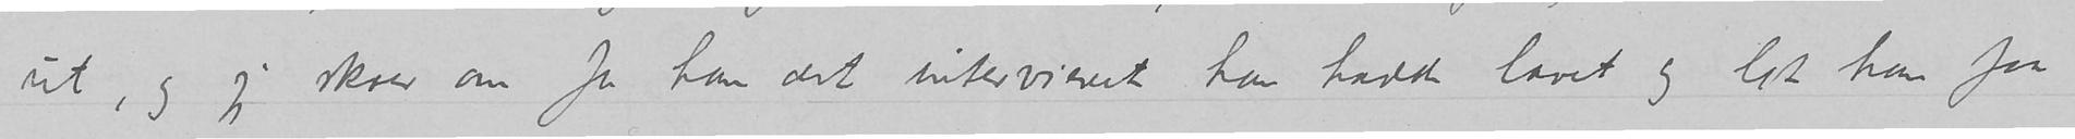ut, og jeg skrev om for han det intervievet han hadde lavet og lot han faa
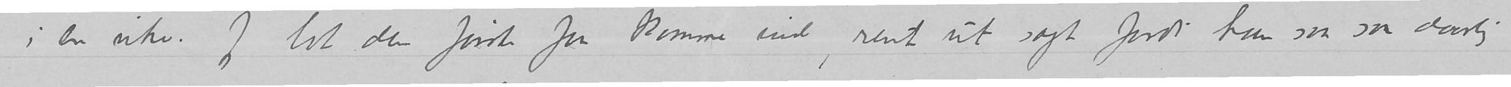i en uke. Jeg lot den første faa komme ind, rent ut sagt fordi han saa saa daarlig
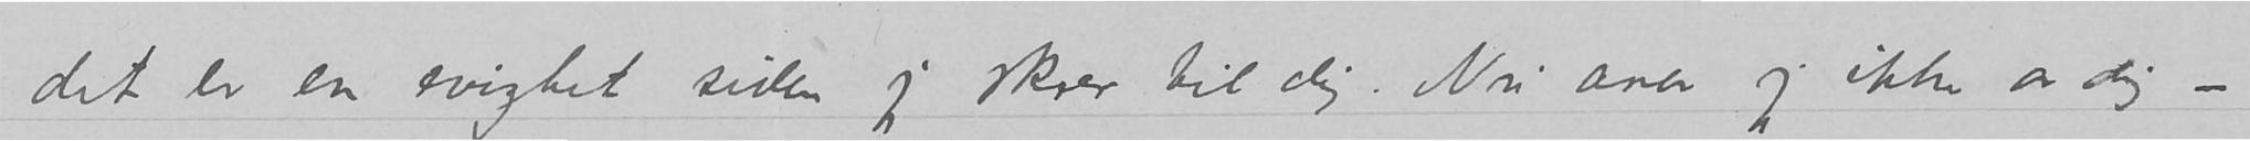det er en evighet siden jeg skrev til dig. Nu aner jeg ikke av dig -
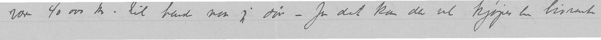være 40 000 kr. til hende naar jeg dør – for det kan der vel kjøpes en livrente
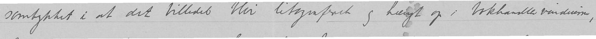samtykket i at det billedet blir litograferet og hængt op i bokhandlervinduerne,
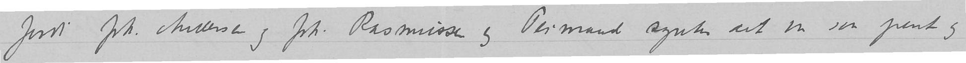fordi frk. Andersen og frk. Rasmussen og Peimand syntes det var saa pent og
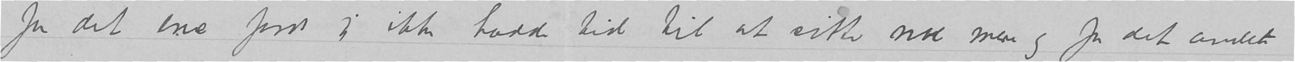for det ene fordi jeg ikke hadde tid til at sitte noe mere og for det andet
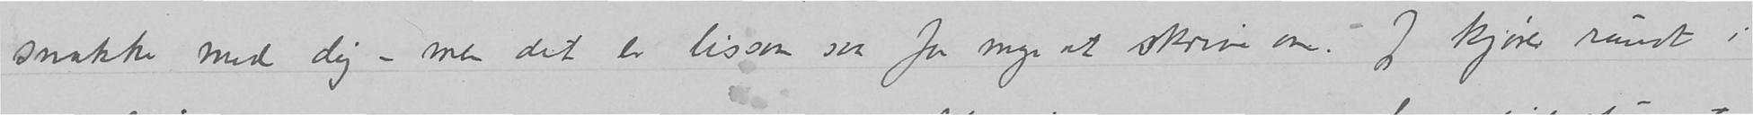snakke med dig – men det er lissom saa for mye at skrive om. Jeg kjører rundt i
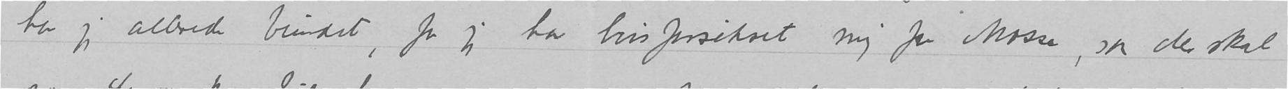har jeg allerede bundet, for jeg har husforsikret mig for Mosse, saa der skal
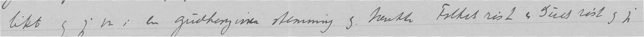likt og jeg var i en gudhengiven stemning og tænkte Folkets røst er Guds røst og jeg
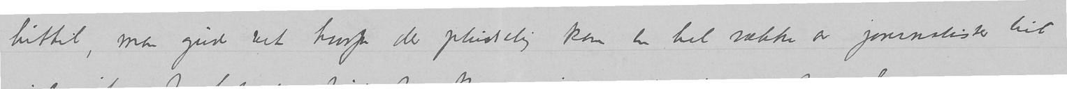hittil, men gud vet hvorfor der pludselig kom en hel række av journalister hit
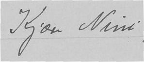Kjære Nini,
